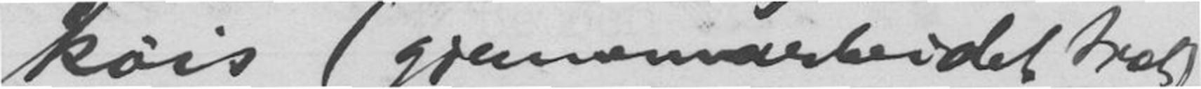køis (gjennemarbeidet træt)
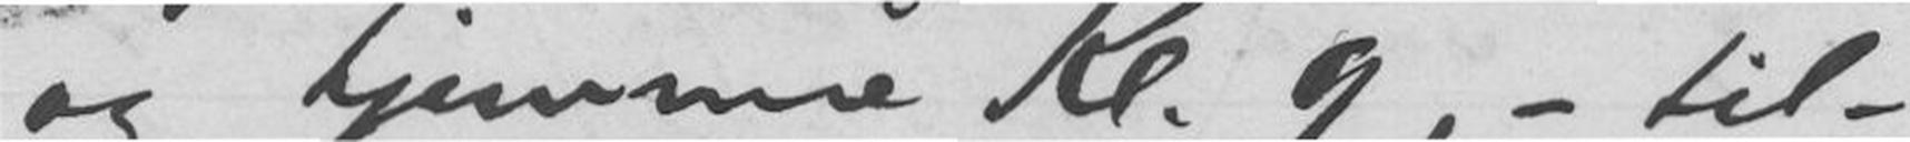og hjemme Kl. 9, - til-
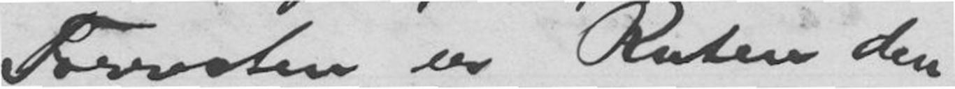Forresten er Ruten den,
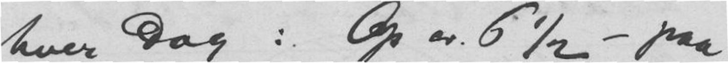hver Dag: Op ca. 6 ½ - paa
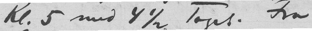Kl. 5 med 4 ½ Toget. Fra
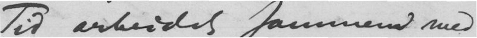Tid arbeidet sammen med
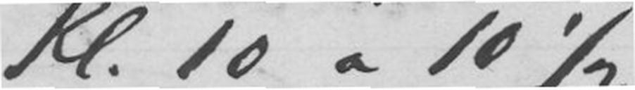Kl. 10 a 10 ½. -
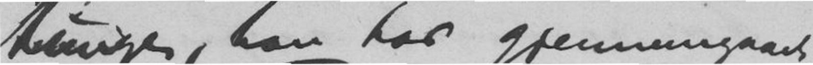tunge, han har gjennemgaaet
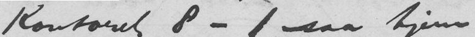Kontoret 8 - 1, saa hjem
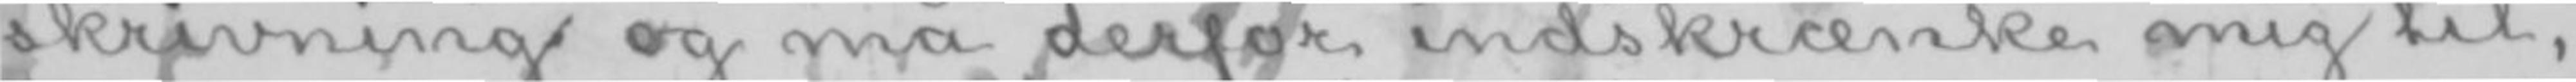skrivning og må derfor indskrænke mig til,
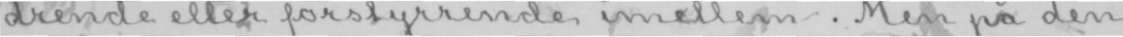drende eller forstyrrende imellem. Men på den
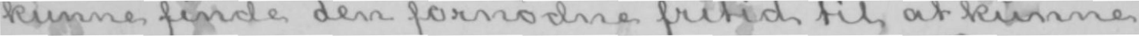kunne finde den fornødne fritid til at kunne
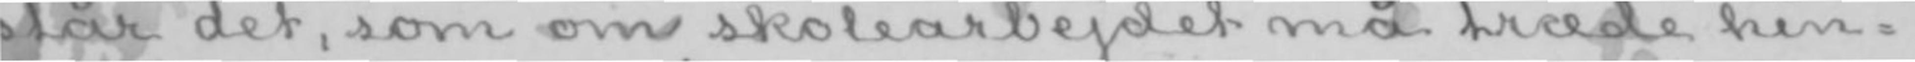står det, som om skolearbejdet må træde hin-
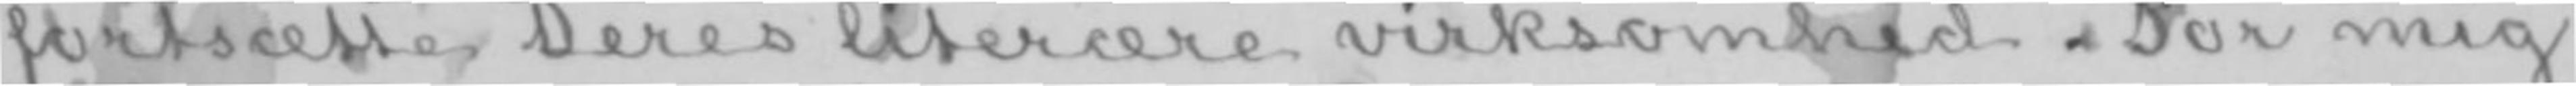fortsætte Deres literære virksomhed. For mig
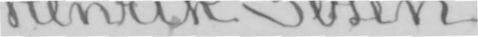Henrik Ibsen.
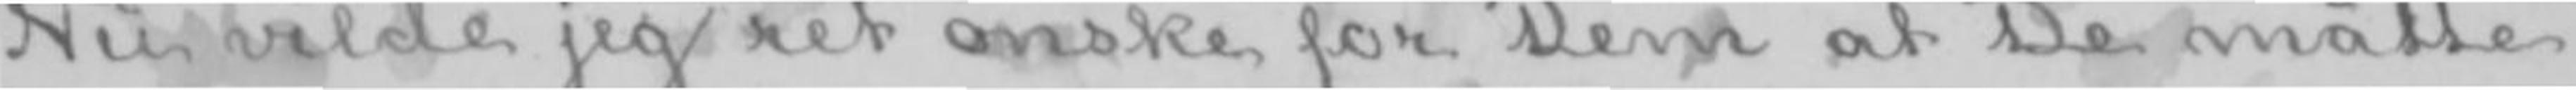Nu vilde jeg ret ønske for Dem at De måtte
